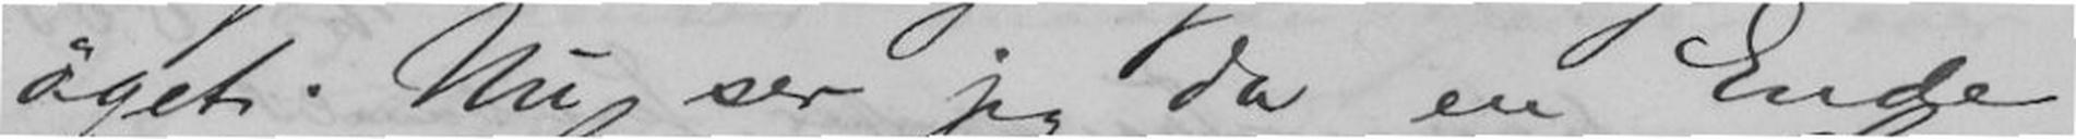øget. Nu ser jeg da en Ende
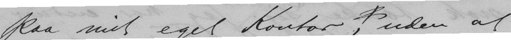paa mit eget Kontor, uden at
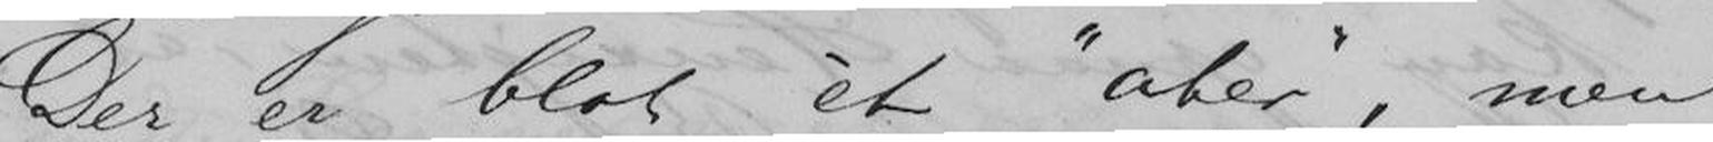Der er blot èt "aber", men
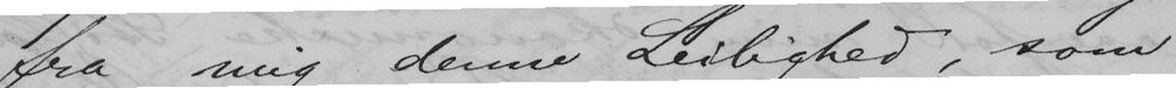fra mig denne Leilighed, som
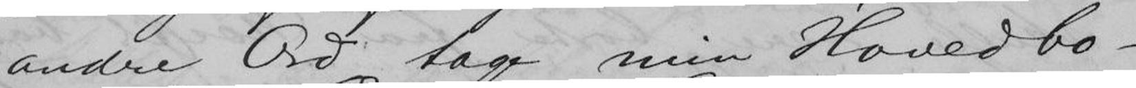andre Ord tage min Hovedbo-
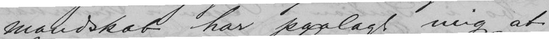mandskab har paalagt mig at
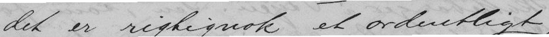det er rigtignok et ordentligt,
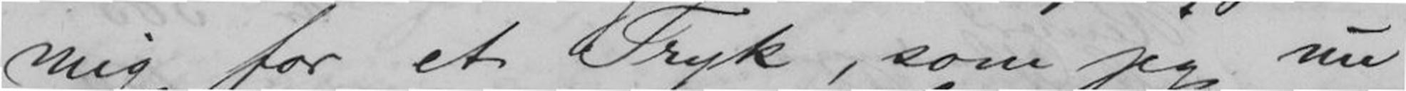mig for et Tryk, som jeg nu
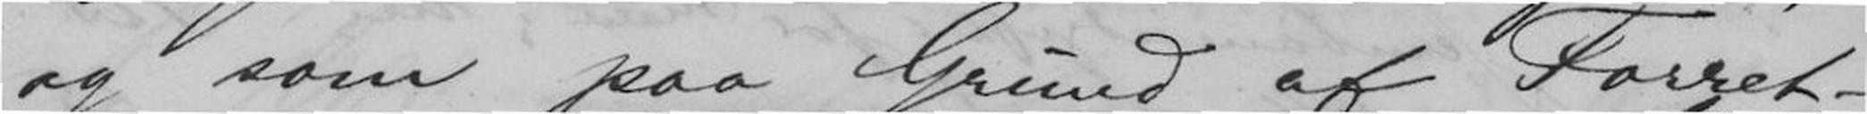og som paa Grund af Forret-
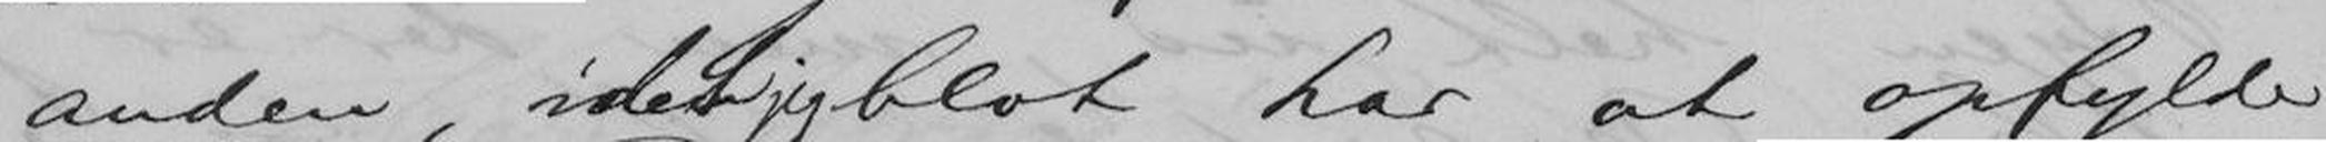anden, idet jeg blot har at opfylde
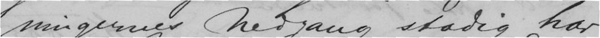ningernes Nedgang stadig har
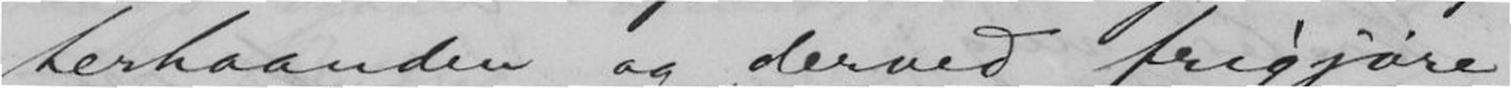terhaanden og derved frigjøre
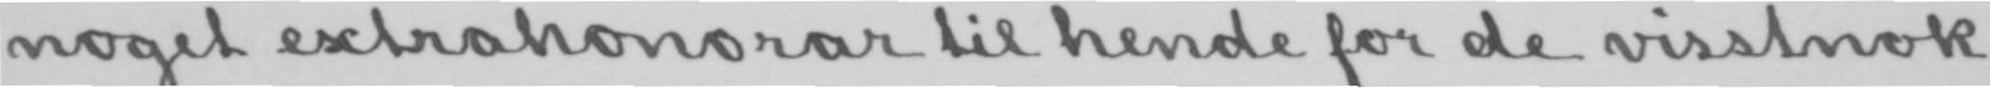noget extrahonorar til hende for de visstnok
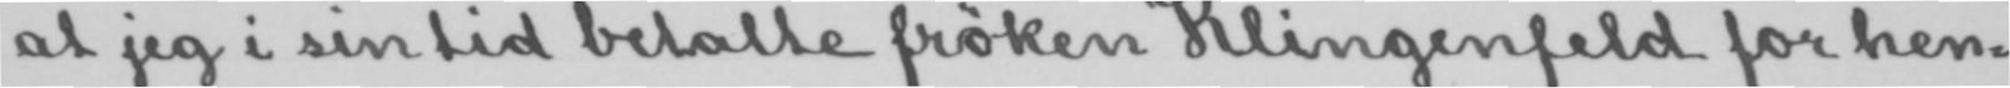at jeg i sin tid betalte frøken Klingenfeld for hen-
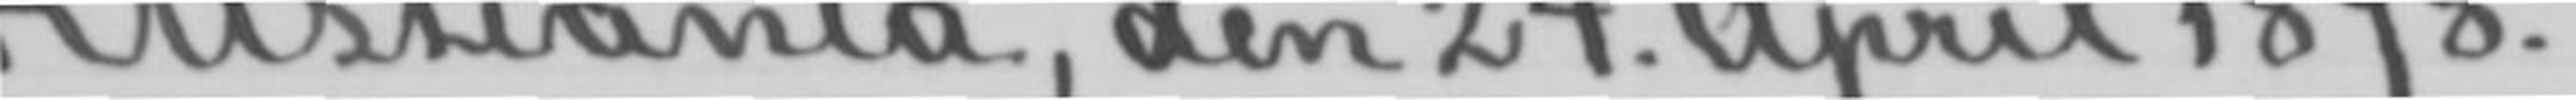Kristiania, den 24. April 1898.
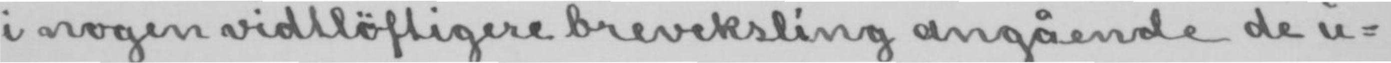i nogen vidtløftigere breveksling angående de u-
